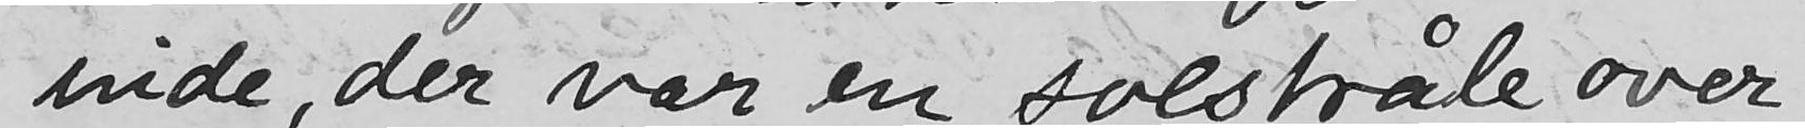inde, der var en solstråle over
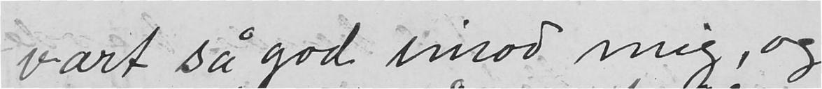vært så god imod mig, og
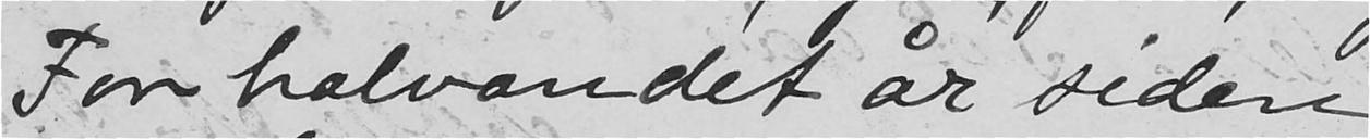For halvandet år siden
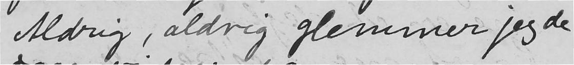Aldrig, aldrig glemmer jeg de
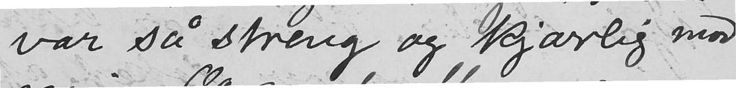var så streng og kjærlig mod
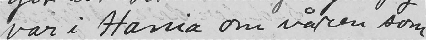var i Xania om våren som
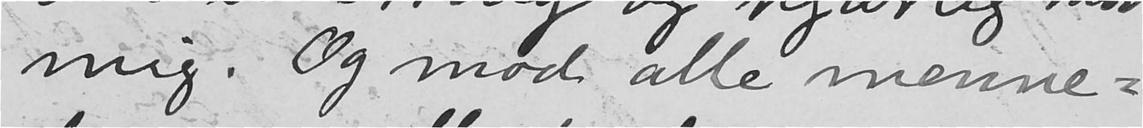mig. Og mod alle menne-
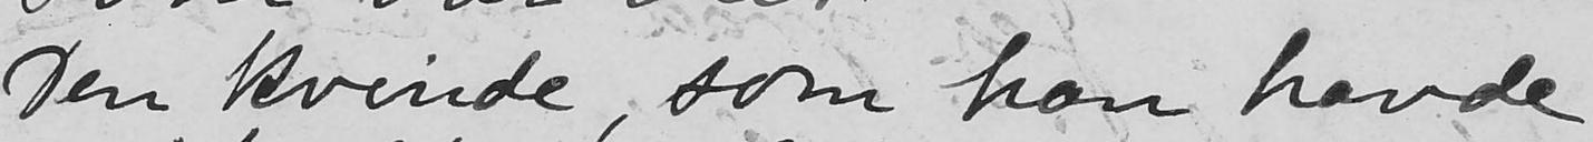Den kvinde, som han havde
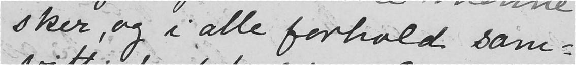sker, og i alle forhold sam-
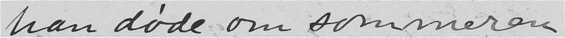han døde om sommeren
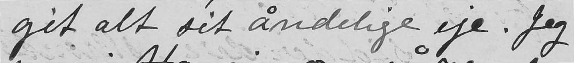git alt sit åndelige eje. Jeg
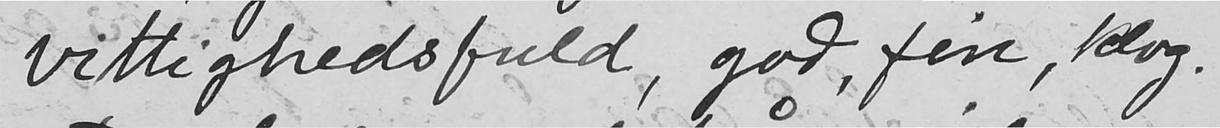vittighedsfuld, god, fin, klog.
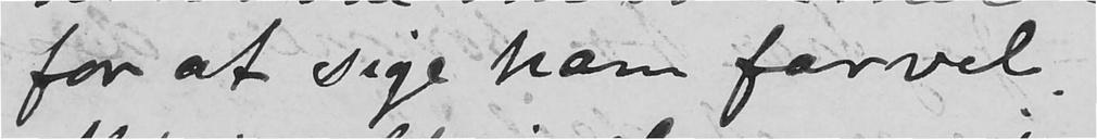for at sige ham farvel.
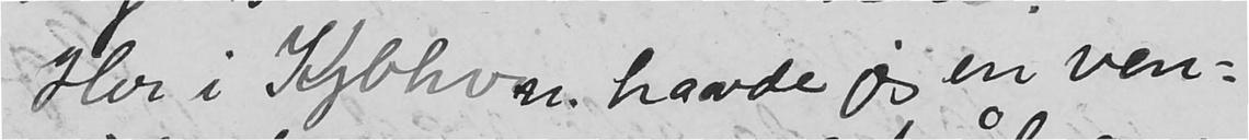Her i Kjbhvn. havde jeg en ven-
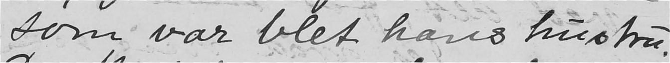som var blet hans hustru.
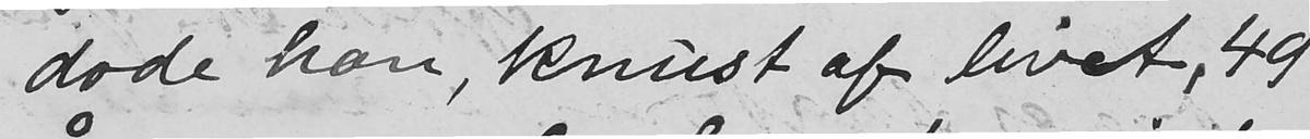døde han, knust af livet, 49
